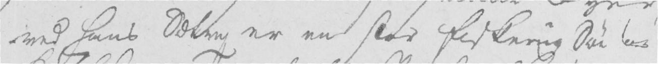ved hans Sæter er en stor fiskerig Søe og
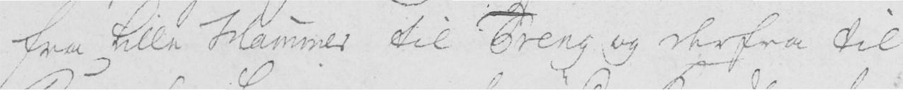fra Lille Hammer til Treng og derfra til
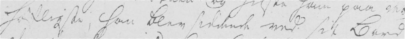høfligste, han blev siddende ved sit Bord
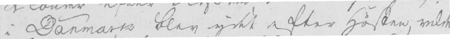i Danmark blev ydet efter Høsten, vilde
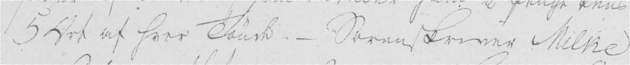5 Ort af hver Tønde. - Sorenskriver Nilse
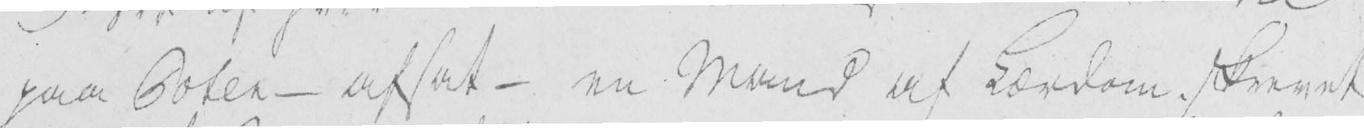paa Toten - afsat - en Mand af Lærdom, skrevet
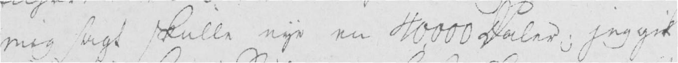mig sagt skulle nye en 40000 Daler; jeg gik
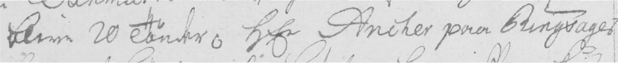blive 20 Tønder; Hr Ancher paa Ringsager
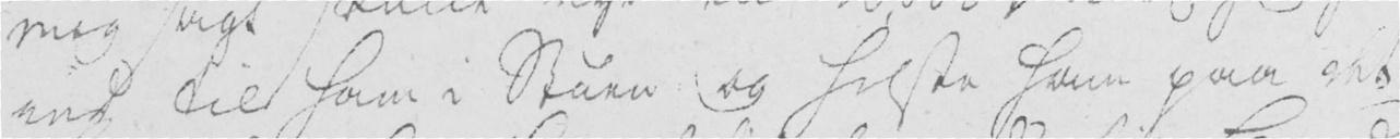ind til ham i Stuen og hilste ham paa det
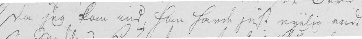da jeg kom ind, han havde just nyelig endt
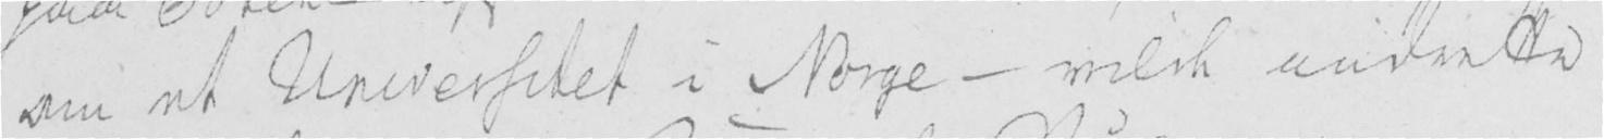om et Universitet i Norge - vilde indrette
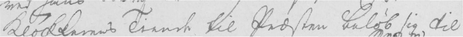Klokkerens Tiende til Præsten anløb sig til
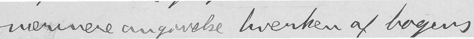nærmere angivelse hverken af bogens
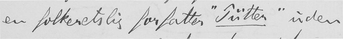en folkeretslig forfatter "Pütter", uden
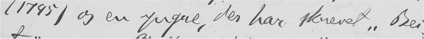(1795) og en yngre, der har skrevet "Bei-
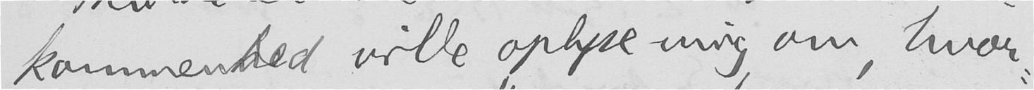kommenhed ville oplyse mig om, hvor-
